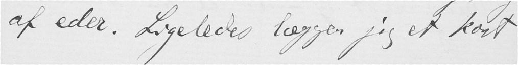af eder. Ligeledes lægger jeg et kort
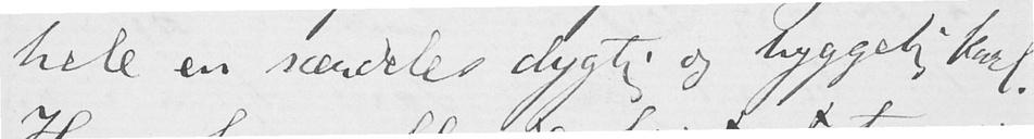hele en særdeles dygtig og hyggelig karl.
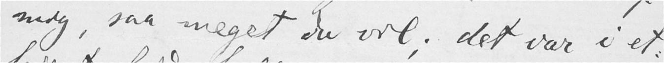mig, saa meget du vil; det var i et-
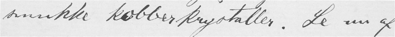smukke kobberkrystaller. Le nu af
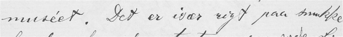muséet. Det er især rigt paa smukke
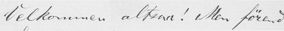Velkommen altsaa! Men føreud
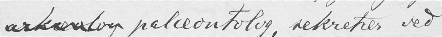arkæolog palæontolog, sekreter ved
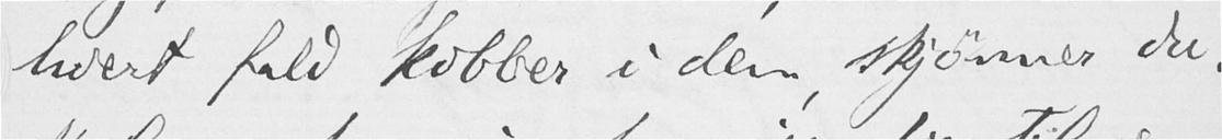hvert fald kobber i dem, skjønner du.
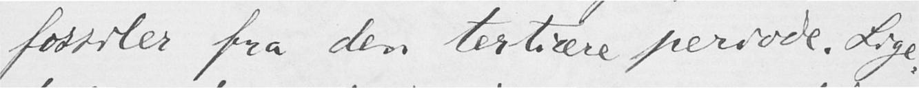fossiler fra den tertiære periode. Lige-
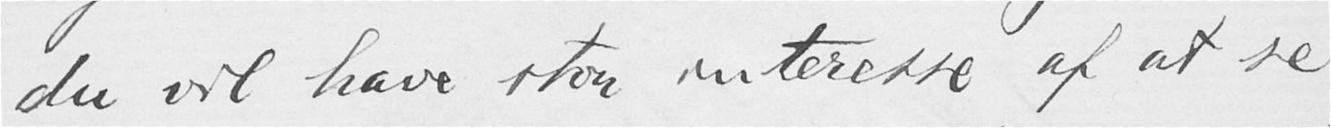du vil have stor interesse af at se
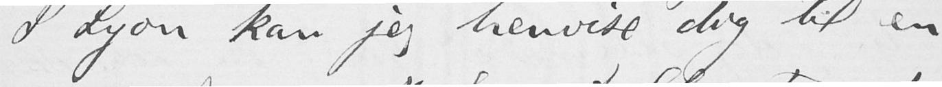I Lyon kan jeg henvise dig til en
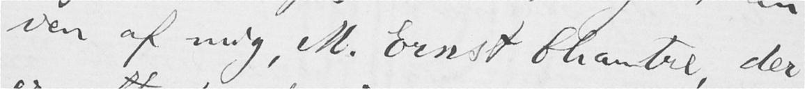ven af mig, M. Ernst Chambre, der
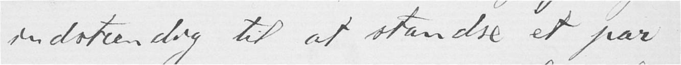indstændig til at standse et par
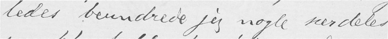ledes beundrede jeg nogle særdeles
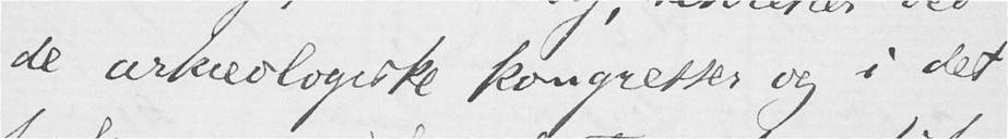de arkæologiske kongresser og i det
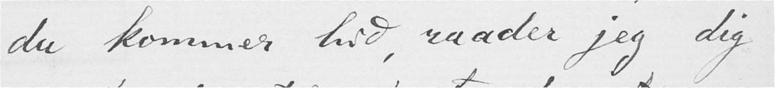du kommer hid, raader jeg dig
